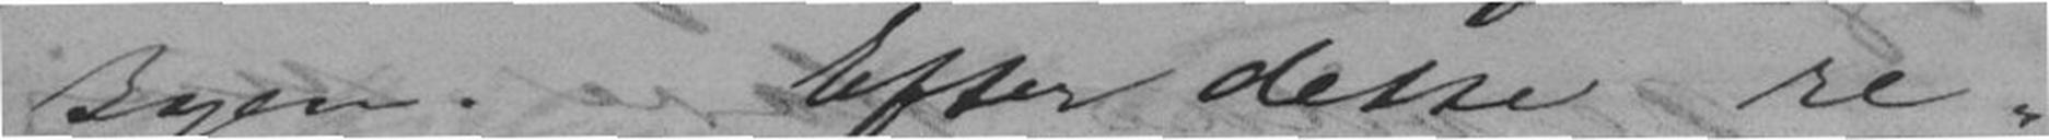Byen. Efter dette re-
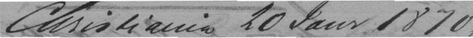Christiania 20 Janr. 1870.
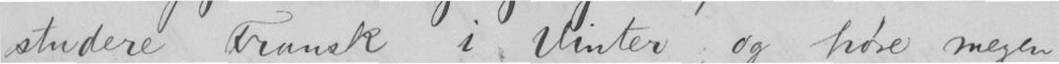studere Fransk i Vinter og høre megen
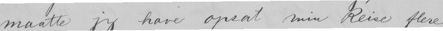maatte jeg have opsat min Reise flere
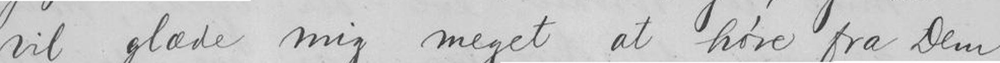vil glæde mig meget at høre fra Dem
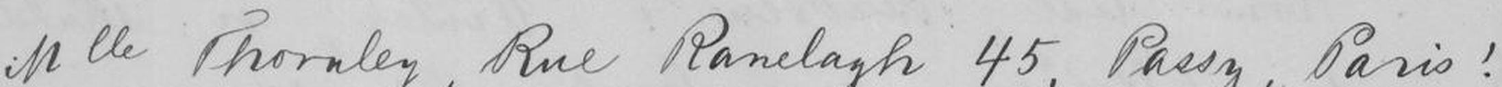Mlle Thornley, Rue Ranelagh 45, Passy, Paris!
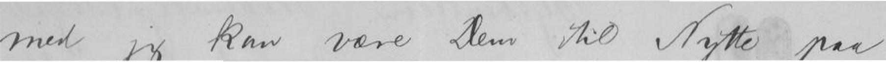med jeg kan være Dem til Nytte paa
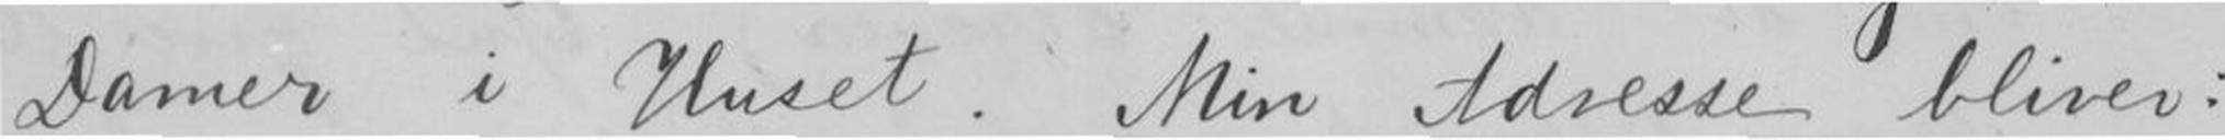Damer i Huset. Min Adresse bliver:
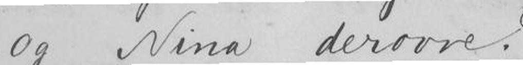og Nina derovre.
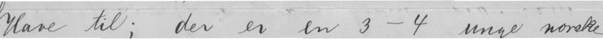Have til; der er en 3-4 unge norske
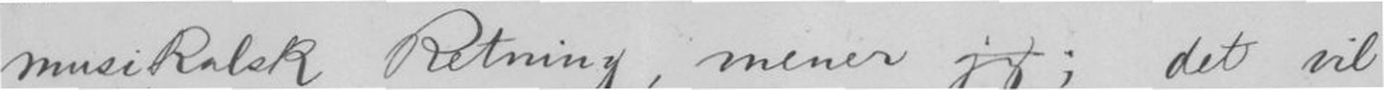musikalsk Retning, mener jeg; det vil
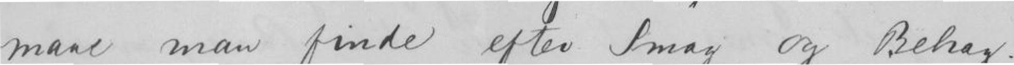maae man finde efter Smag og Behag.
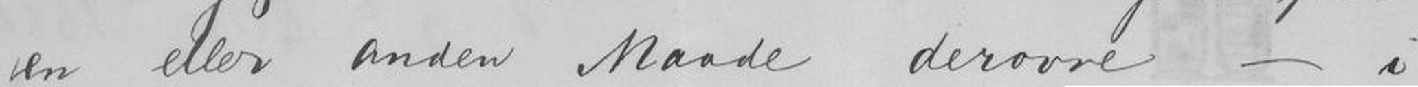en eller anden Maade derovre - i
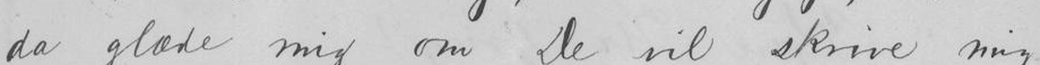da glæde mig om De vil skrive mig
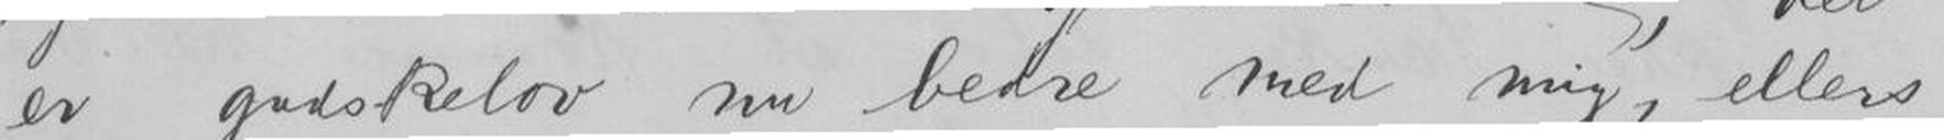er gudskelov nu bedre med mig, ellers
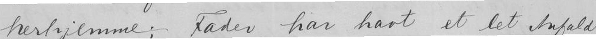herhjemme; Fader har havt et let Anfald
